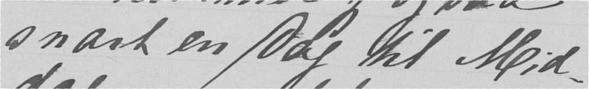snart en Dag til Mid-
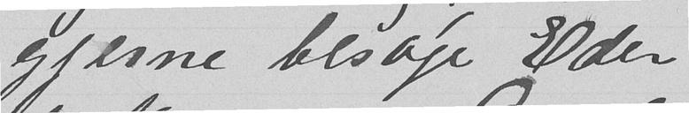gjerne besøge Eder
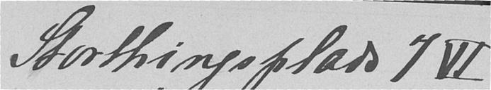Storthingsplads 7VI
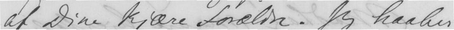af Dine kjære Forældre. Jeg haaber
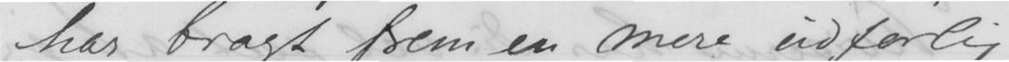har bragt frem en mere udførlig
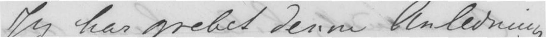Jeg har grebet denne Anledning
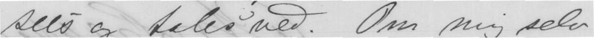seés og tales ved. Om mig selv
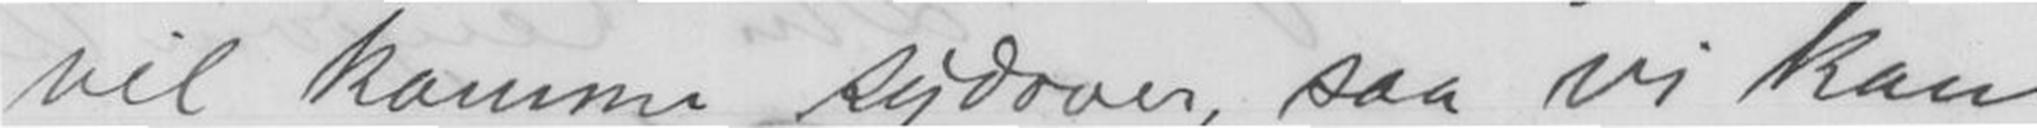vil komme sydover, saa vi kan
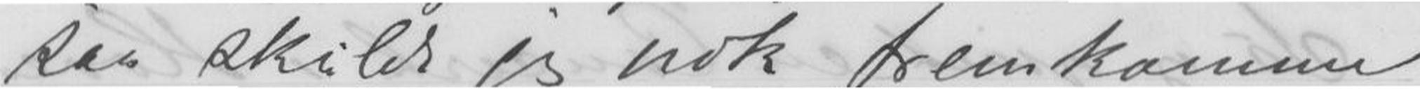saa skulde jeg nok fremkomme
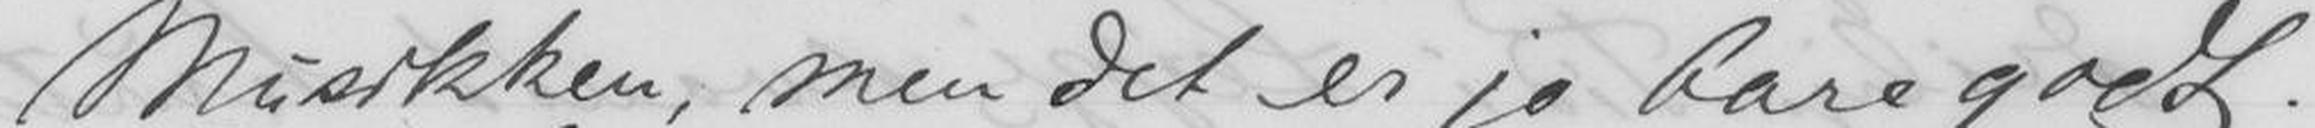Musikken, men det er jo bare godt.
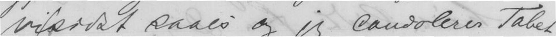vi sidst saaes og jeg Condolerer Tabet
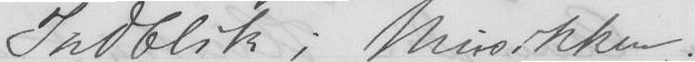Indblik i Musikken.
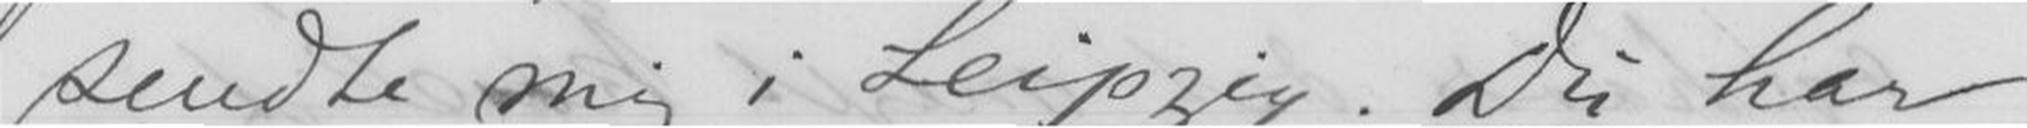sendte mig i Leipzig. Du har
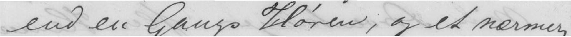end en Gangs Høren. og et nærmere
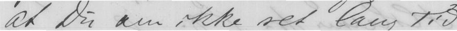at Du om ikke ret lang Tid
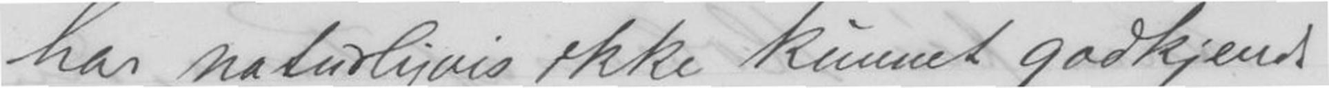har naturligvis ikke kunnet godkjende
